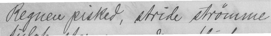Regnen pisked, stride strømme
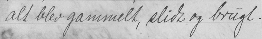alt blev gammelt, slidt og brugt.
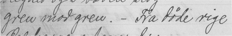gren mod gren. - Fra døde rige
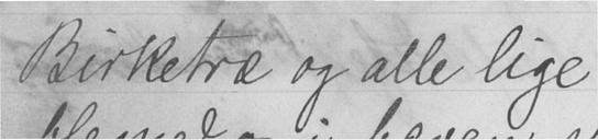Birketræ og alle lige
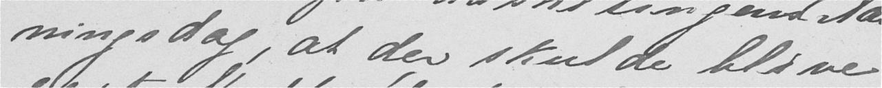ningsdag, at der skulde blive
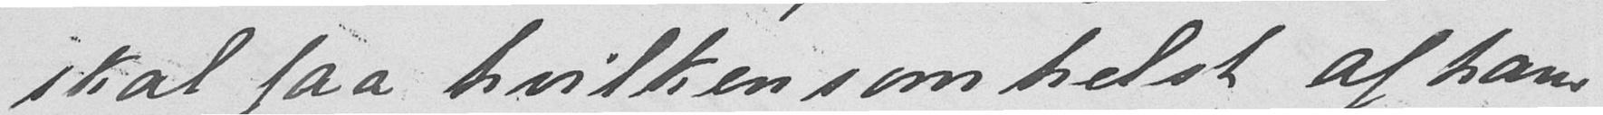skal faa hvilken som helst af hans
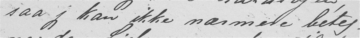saa jeg kan ikke nærmere beteg-
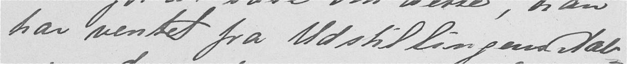har ventet fra Udstillingens Aab-
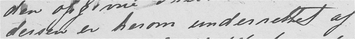dersen er herom underrettet af
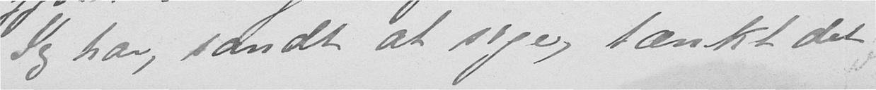Jeg har, sandt at sige, tænkt det
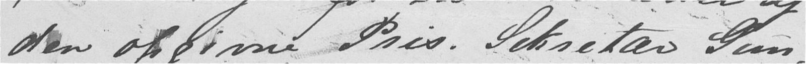den opgivne Pris. Sekretær Gun-
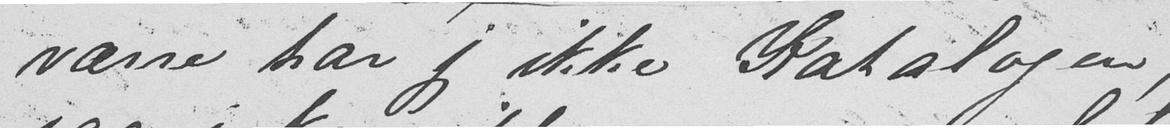værre har jeg ikke Katalogen,
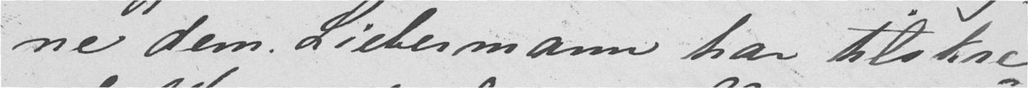ne dem. Liebermann har tilskre-
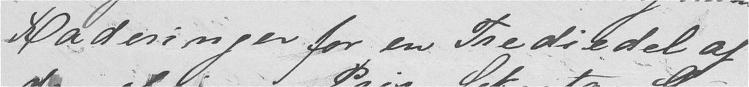Raderinger for en Trediedel af
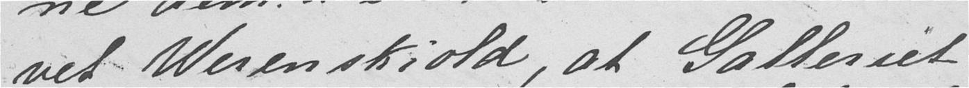vet Werenskiold, at Galleriet
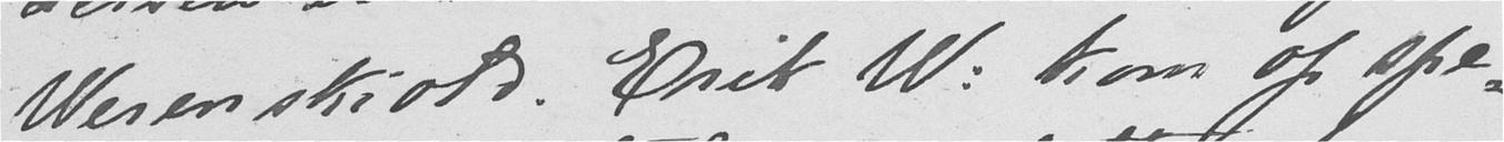Werenskiold. Erik W. kom op spe-
